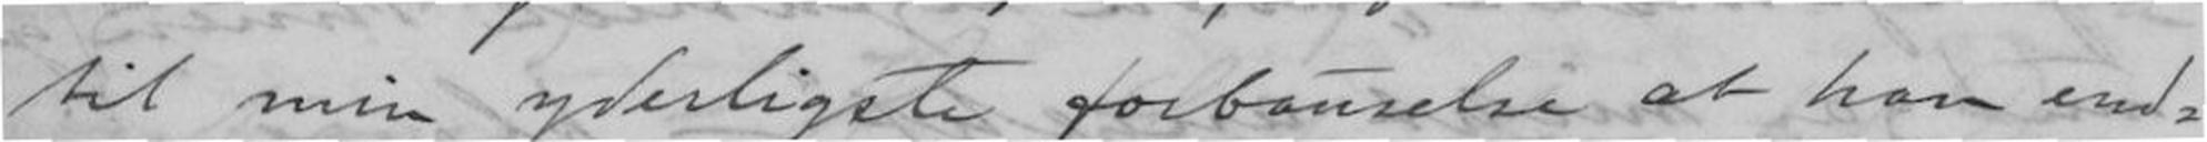til min yderligste forbauselse at han end-
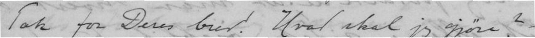Tak for Deres Brev! Hvad skal jeg gjøre? -
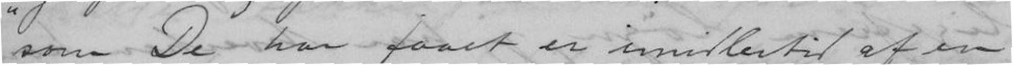som De har faaet er imidlertid af en
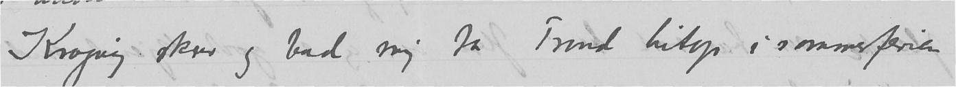Krogvig skrev og bad mig ta Trond hitop i sommerferien
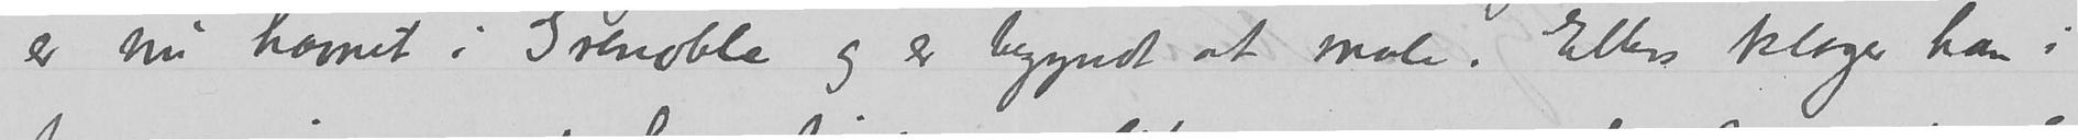er nu havnet i Grenoble og er begyndt at male. Ellers klager han i
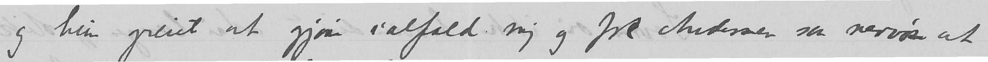og hun greiet at gjøre ialfald mig og frk Andersen saa nervøse at vi
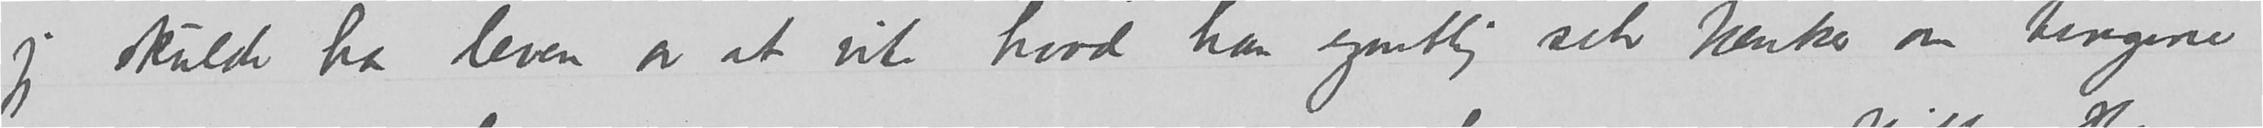jeg skulde ha leven av at vite hvad han egentlig selv tænker om tingene.
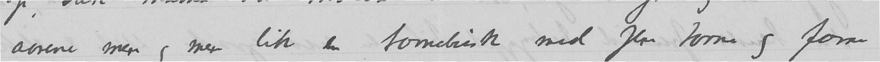aarene mere og mere lik en tornebusk med flere torner og færre
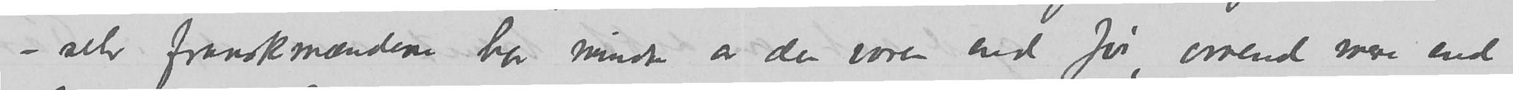– selv franskmændene har mindre av den varen end før, omend mere end
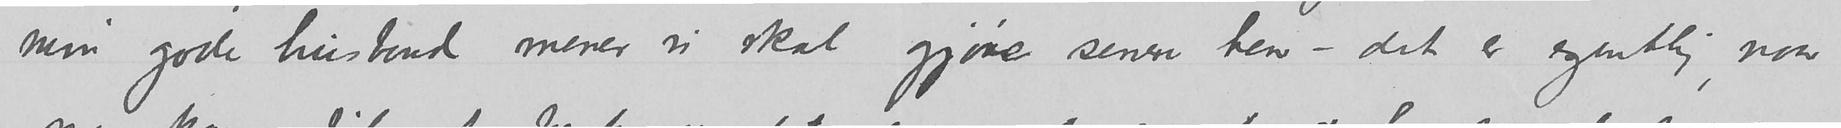min gode husbond mener vi skal gjøre senere hen – det er egentlig, naar
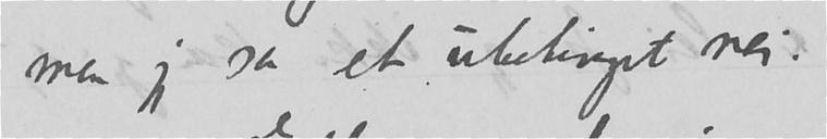men jeg sa et ubetinget nei.
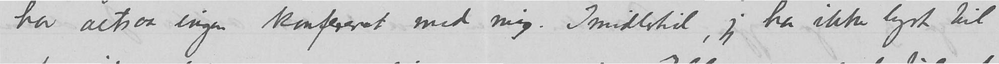har altsaa ingen konfereret med mig.  Imidlertid, jeg har ikke lyst til
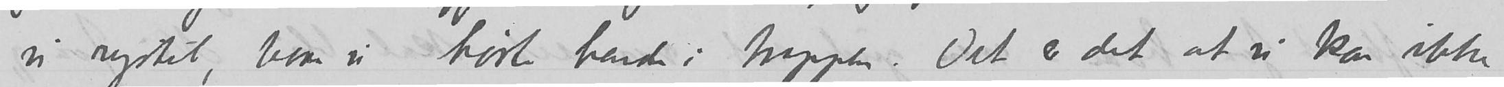rystet, bare vi hørte hende i trappen. Det er det at vi kan ikke
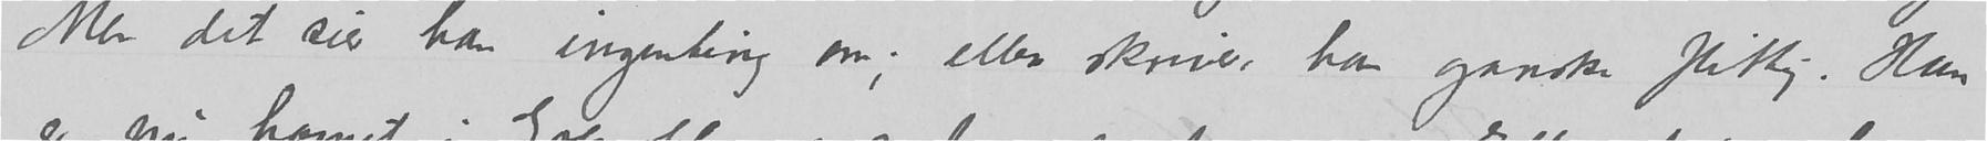Men det sier han ingenting om; ellers skriver han ganske flittig. Han
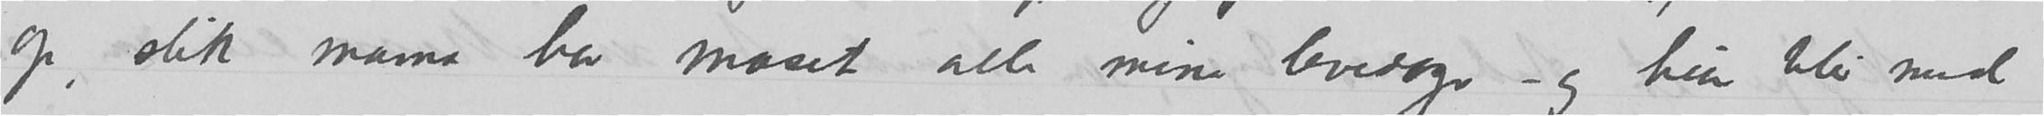op, slik mama har maset alle mine levedager – og hun blir med
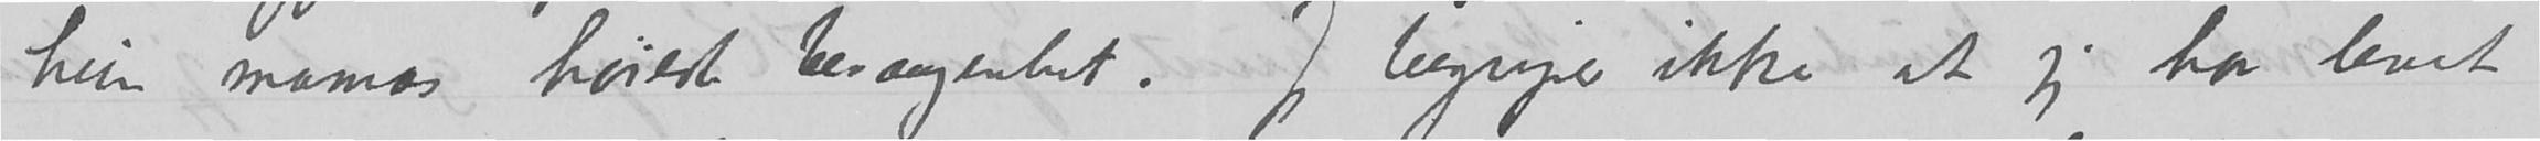hun mamas høieste bevaagenhet. Jeg begriper ikke at jeg har levet
